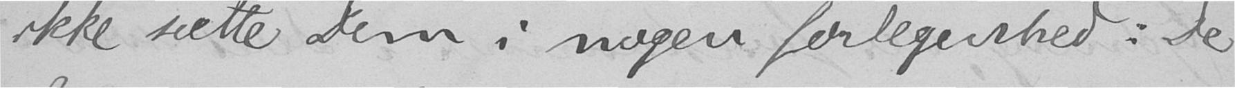ikke sætte Dem i nogen forlegenhed: De
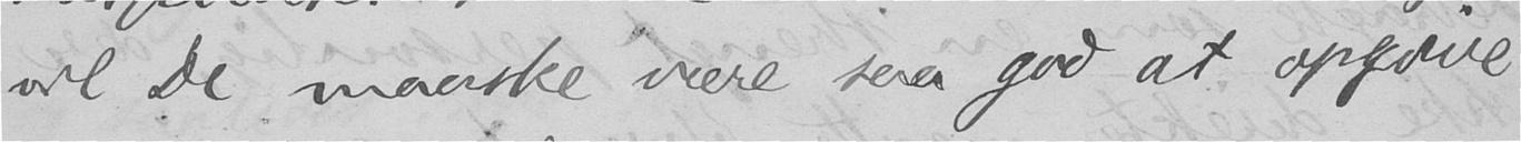vil De maaske være saa god at opgive
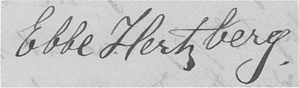Ebbe Hertzberg.
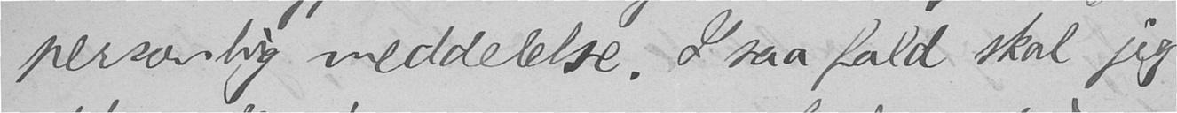personlig meddelelse. I saa fald skal jeg
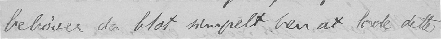behøver da blot simpelt hen at lade dette
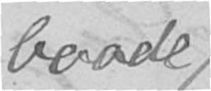baade
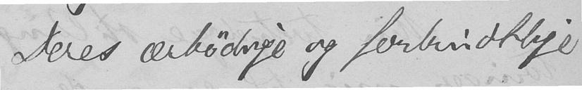Deres ærbødige og forbindtlige
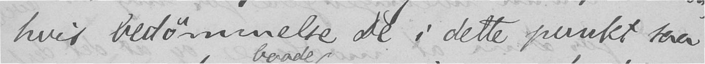hvis bedømmelse De i dette punkt saa
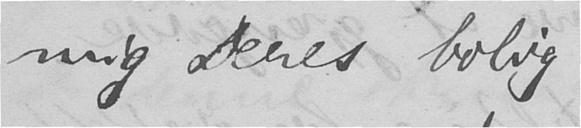mig Deres bolig.
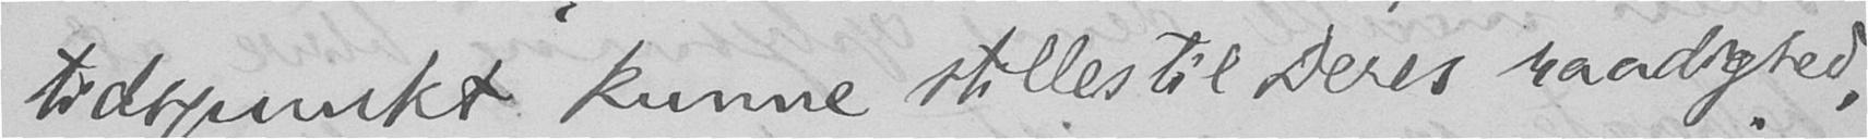tidspunkt kunne stilles til Deres raadighed,
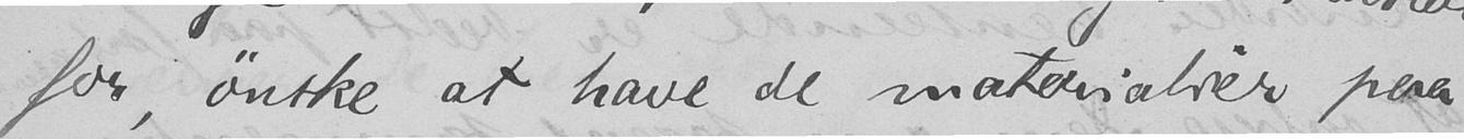for, ønske at have de materialier paa
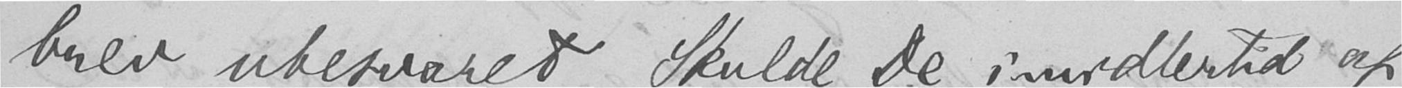brev ubesvaret. Skulde De imidlertid af
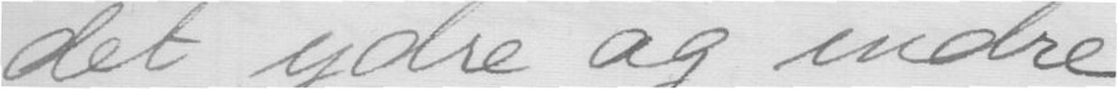det ydre og indre.
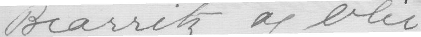Biarritz og blir
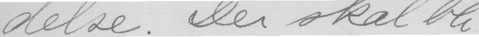delse. Der skal bli
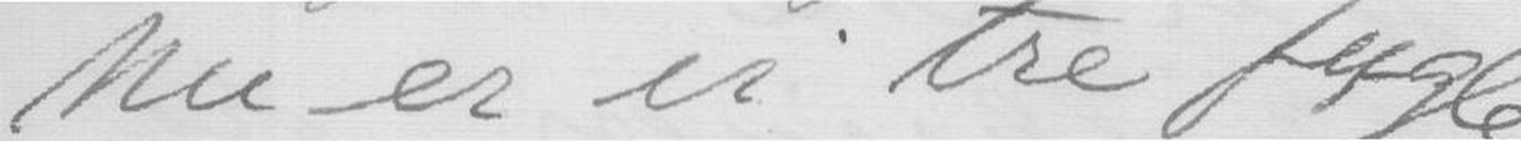Nu er vi tre fugle
